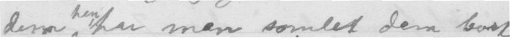dem hen, har man somlet dem bort
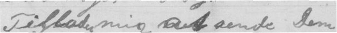Tillader mig at sende Dem
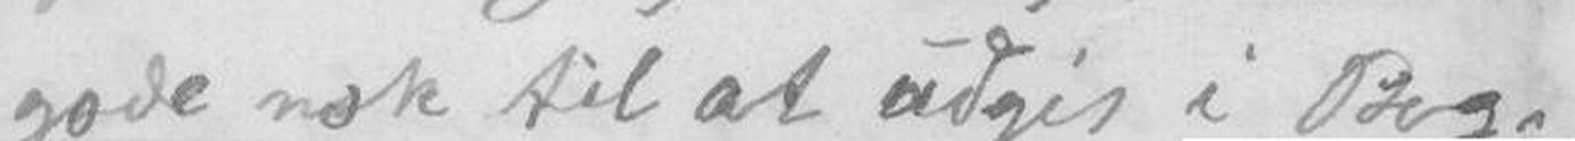gode nok til at udgis i Bog.
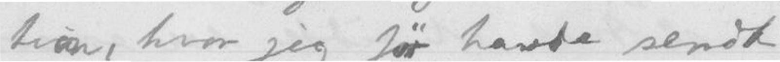tion, hvor jeg før havde sendt
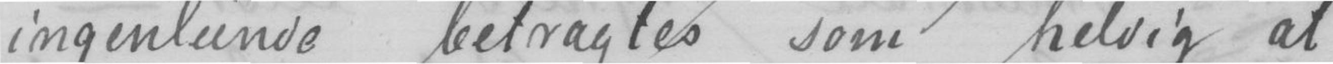ingenlunde betragtes som heldig at
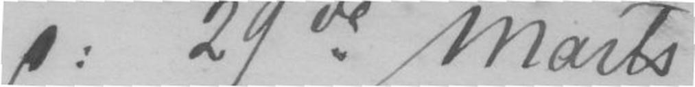D. 29de Marts
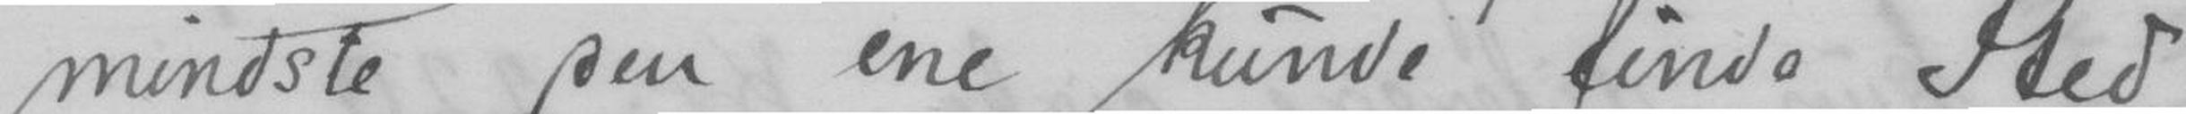mindste den ene kunde finde Sted
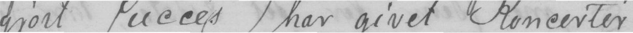gjort Succès har givet Koncerter
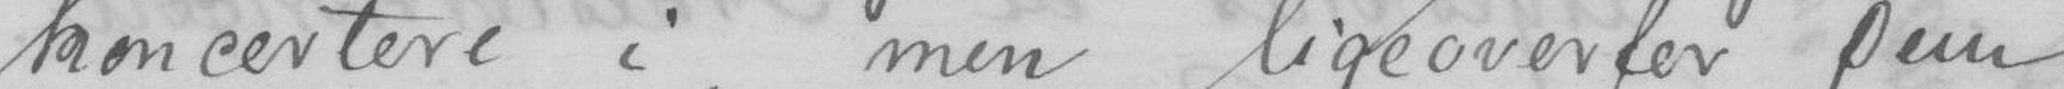koncertere i, men ligeoverfor Dem
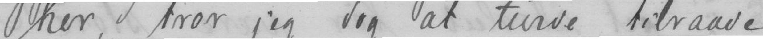her, tror jeg dog at turde tilraade
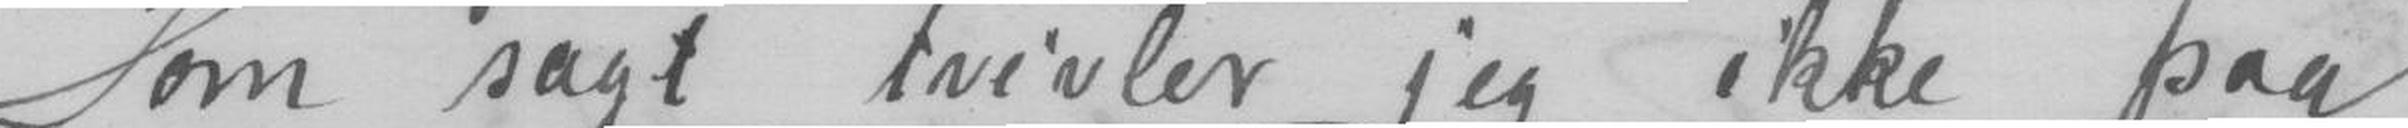Som sagt tvivler jeg ikke paa
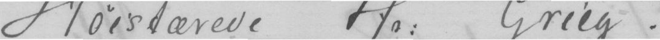Høistærede Hr. Grieg!
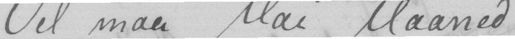Vel maa Mai Maaned
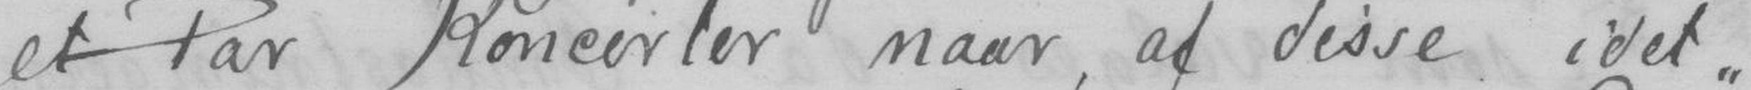et Par Koncerter naar, af disse idet-
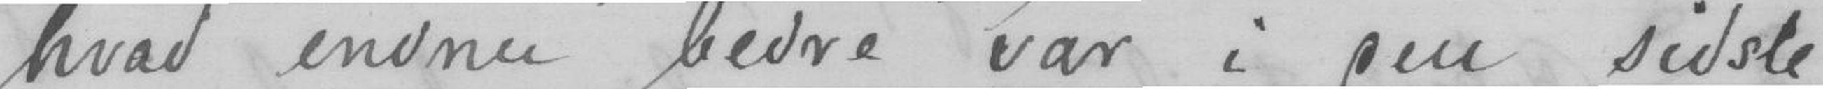hvad endnu bedre var i den sidste
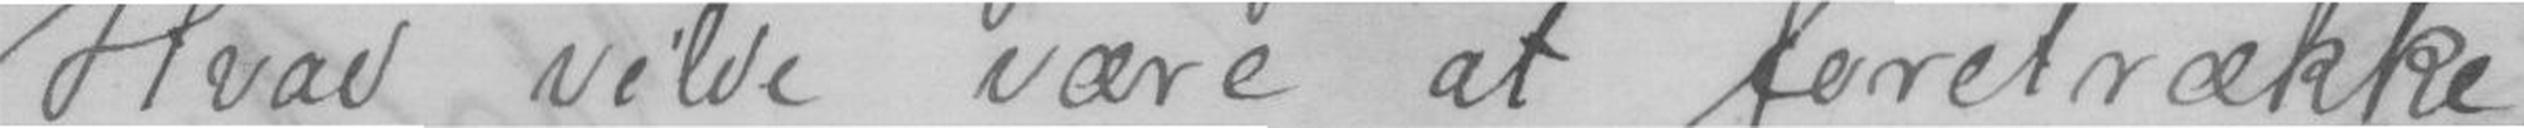Hvad vilde være at foretrække
